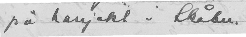på harejakt i Skåben
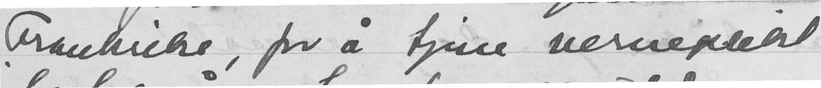Frankrike, for å tjene verneplikt
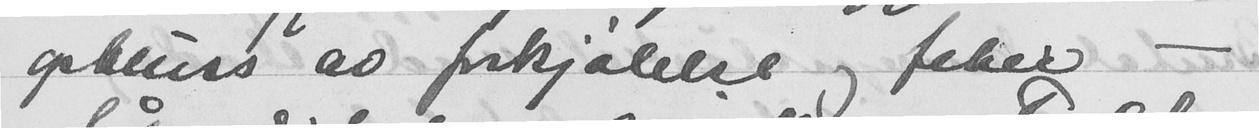opbluss av forkjølelse og feber -
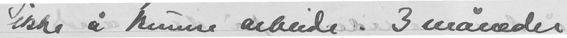ikke å kunne arbeide. 3 måneder
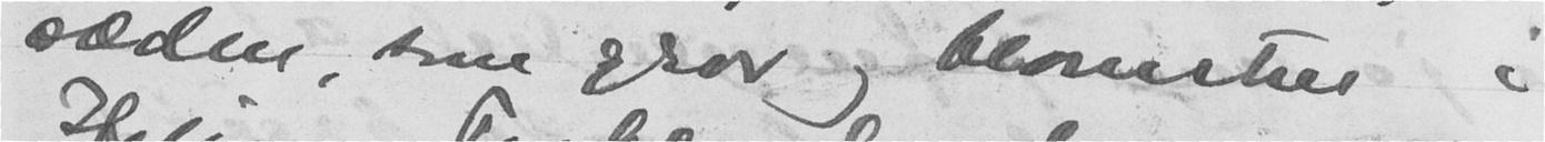sæden, som gror og Homstin i
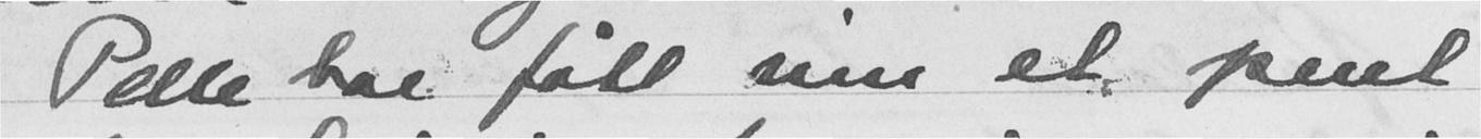Pelle har fått inn et pent
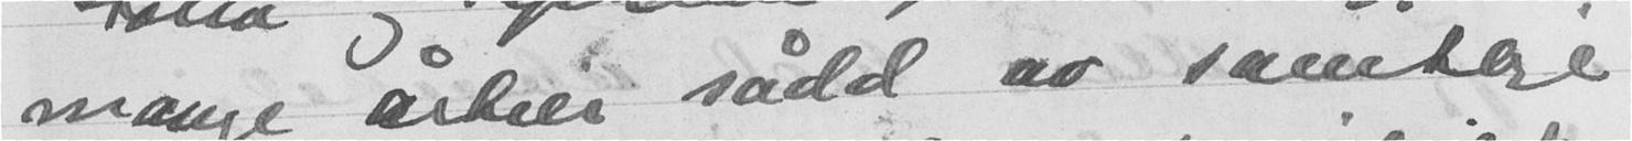mange årtier sådd av samtlige
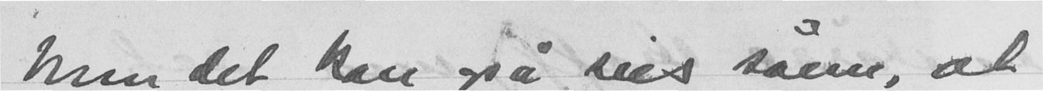Men det kan også sies sånn, at
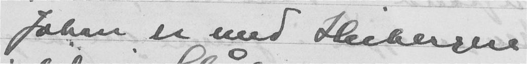Johan er med Heibergese
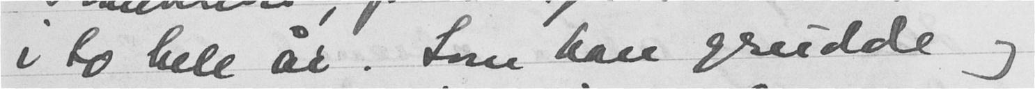i to hele år. Som han grudde og
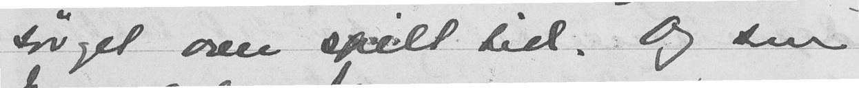sørget over spilt tid. Og som
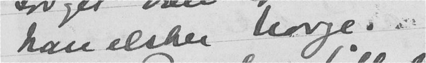han elsker Norge.
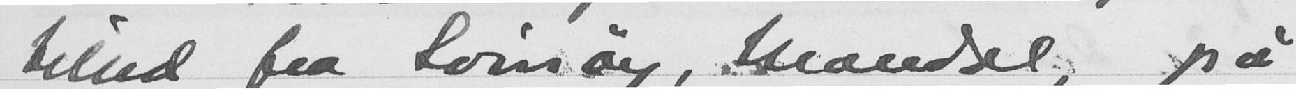billed fra Somay, ,Frandal, på
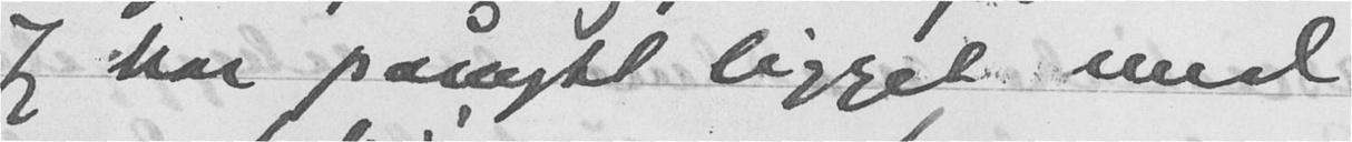Jeg har pånytt ligget med
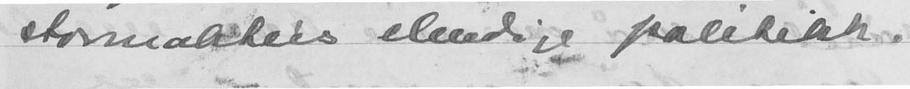stormakters elendige politikk.
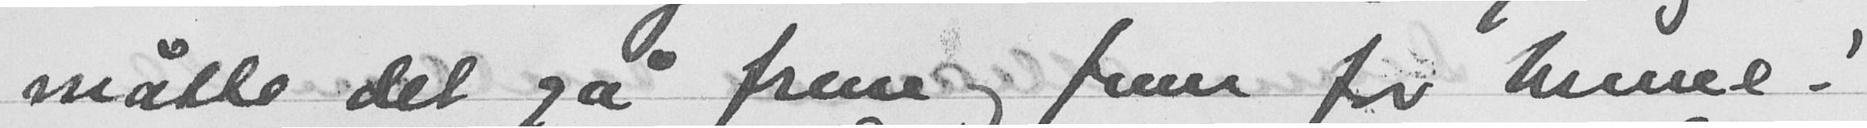måtte det gå frem og frem for henne!
